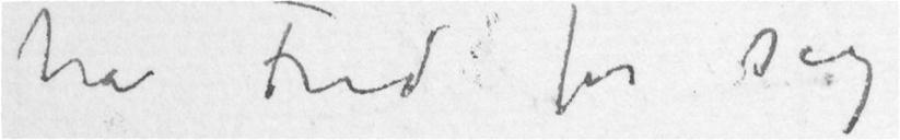ha Fred for sig
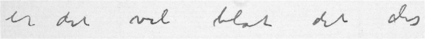er det vel blot det der
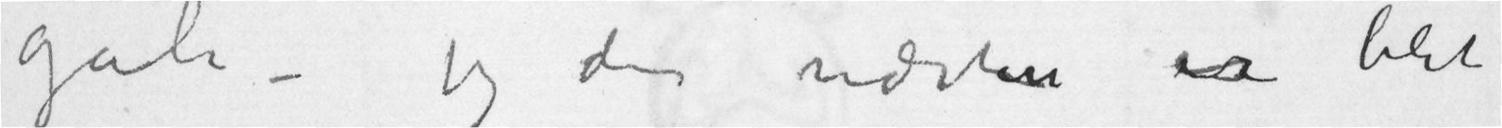gale – jeg der næsten er blit
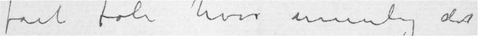fået føle hvor umulig det
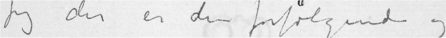jeg der er den forfølgende og
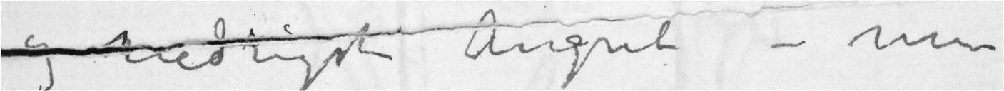og nedrigste Angreb – men
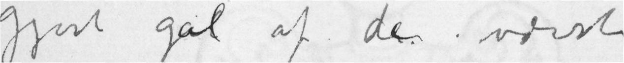gjort gal af de værste
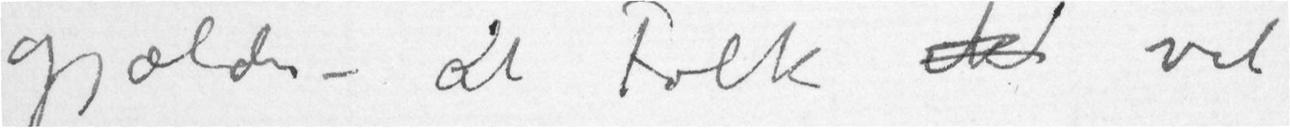gjælder – at Folk ik vil
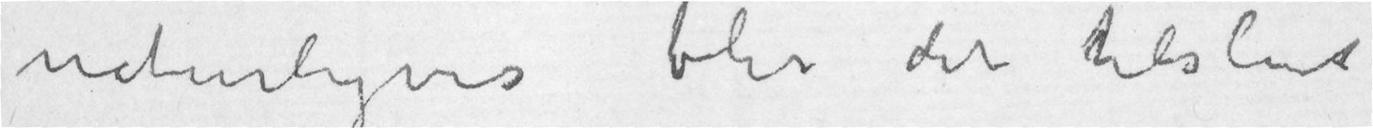naturligvis blir det tilslut
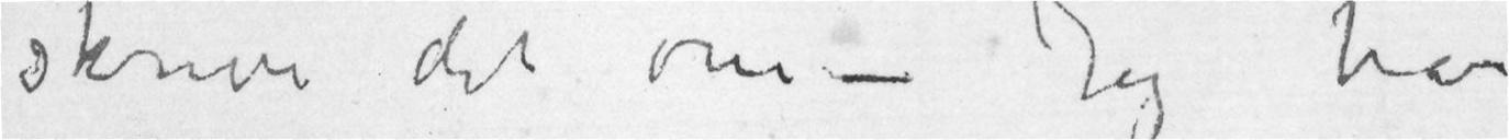skrive det om – Jeg har
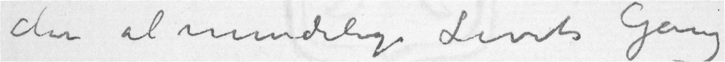den almindelige Livets Gang
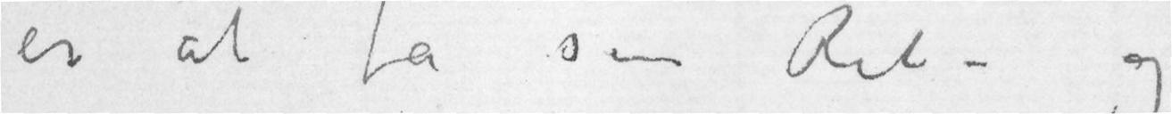er at få sin Ret – og
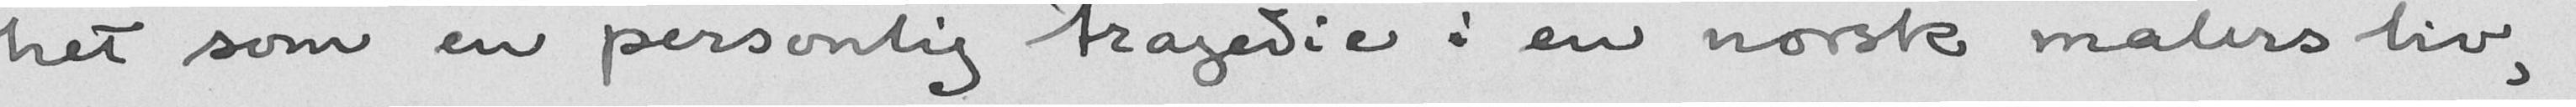het som en personlig tragedie i en norsk malers liv,
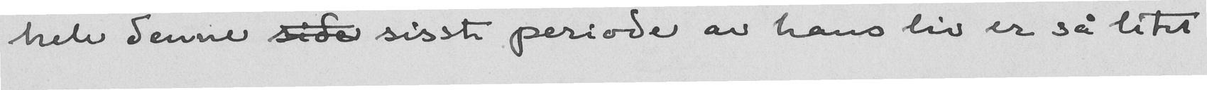hele denne side sisste periode av hans liv er så litet
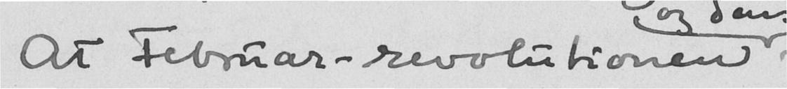At februar-revolutionen
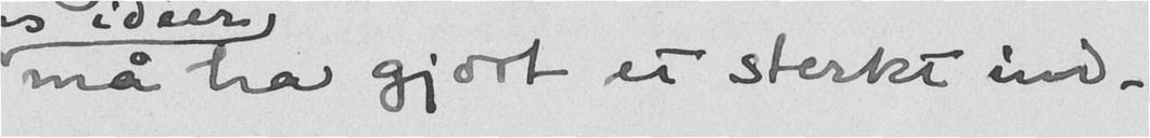må ha gjort et sterkt ind-
